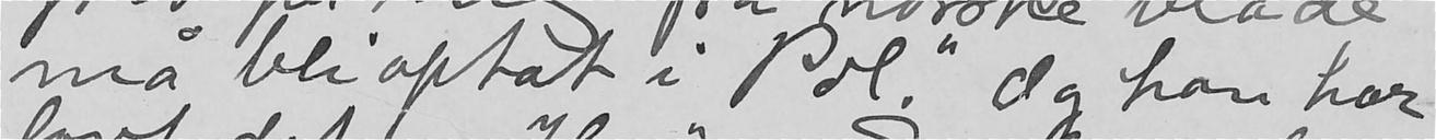må bli optat i "Pol." Og han har
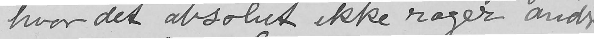hvor det absolut ikke rager andre
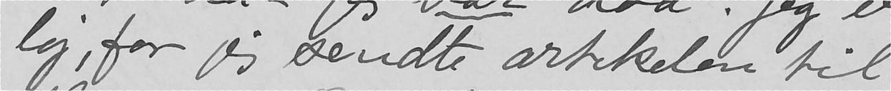lej, for jeg sendte artikelen til
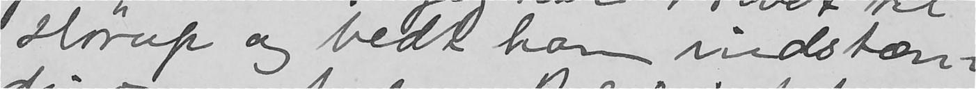Hørup og bedt han indstæn-
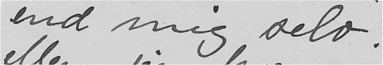end mig selv.
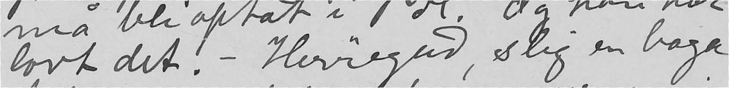lovt det! - Herregud, slig en baga
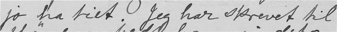jo ha tiet. Jeg har skrevet til
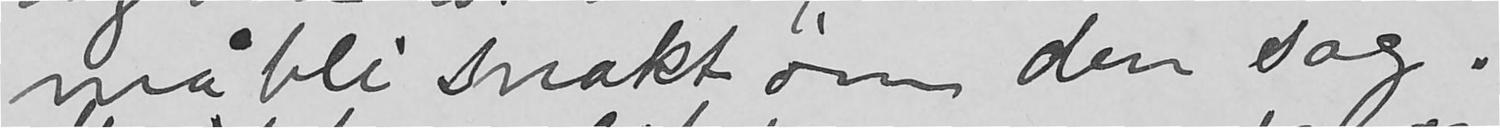må bli snakt om den sag.
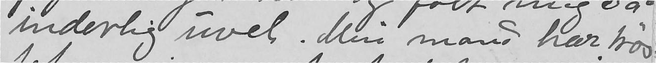inderlig uvel. Min mand har trøs
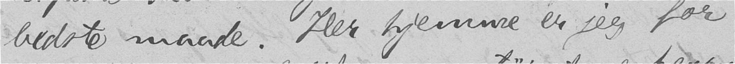bedste maade. Her hjemme er jeg for
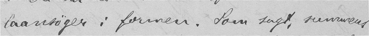laansøger i formen. Som sagt, summens
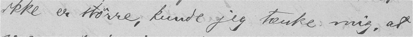ikke er større, kunde jeg tænke mig, at
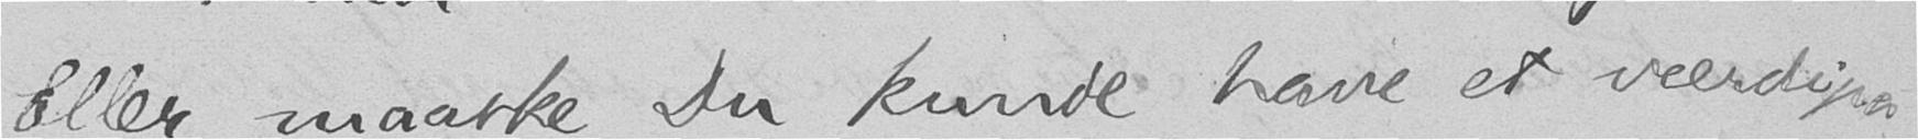Eller maaske Du kunde have et værdipa-
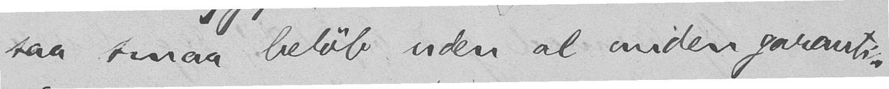saa smaa beløb uden al anden garanti.
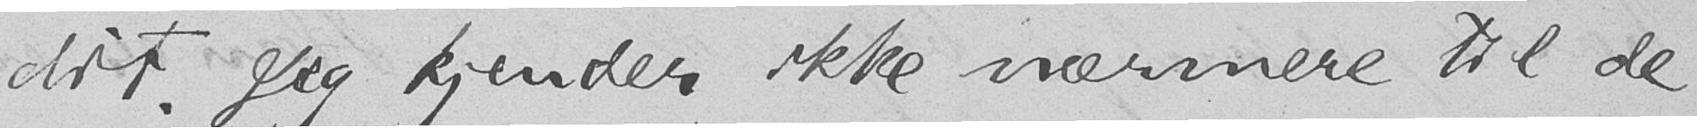dit. Jeg kjender ikke nærmere til de
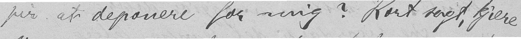pir at deponere for mig? Kort sagt, kjære
